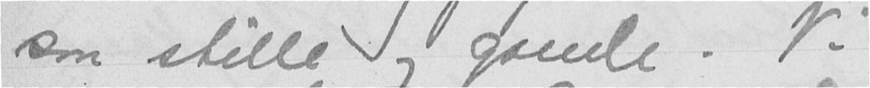saa stille og gamle. Vi
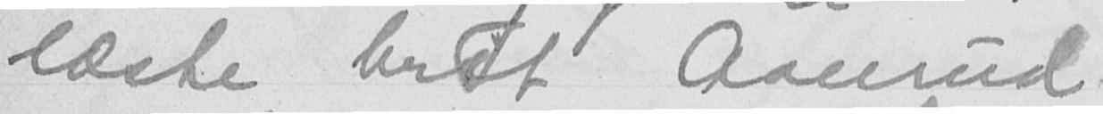læste høit Aanrud
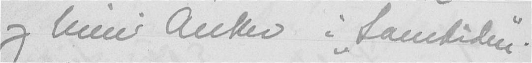og Nini Anker i "Samtiden".
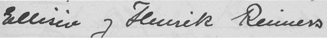Ellisiv og Henrik Reimers
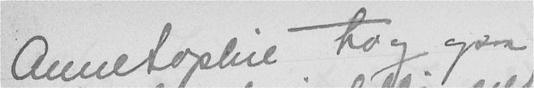Anne Sophie tog ogsaa
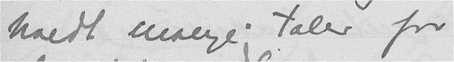holdt mange taler for
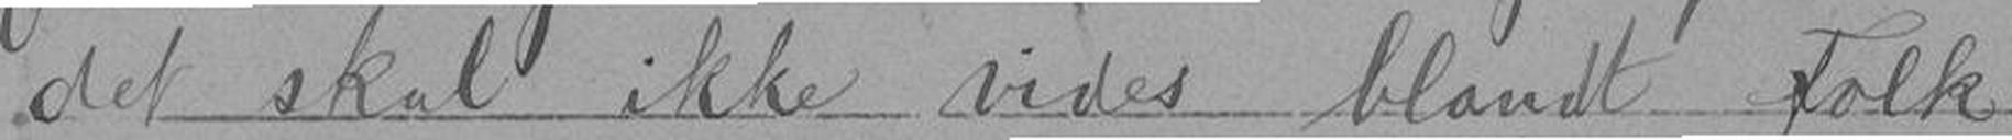det skal ikke vides blandt Folk
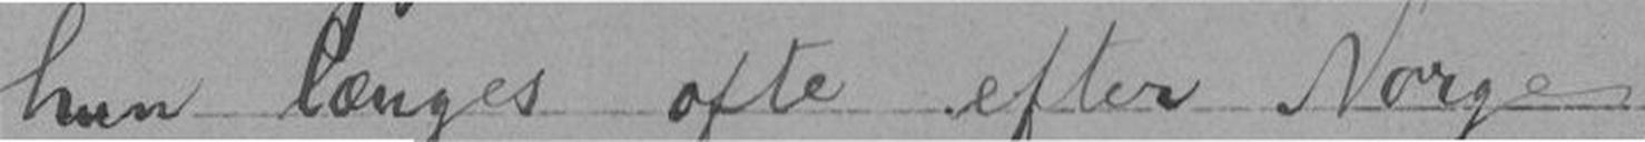hun længes ofte efter Norge,
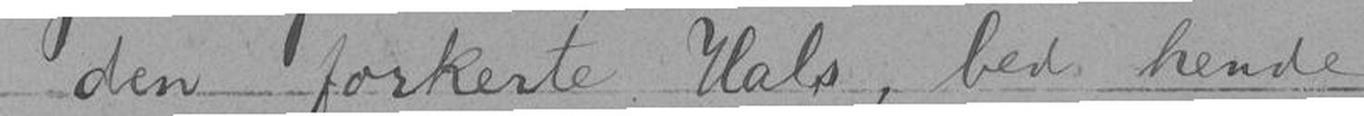den forkerte Hals, bed hende
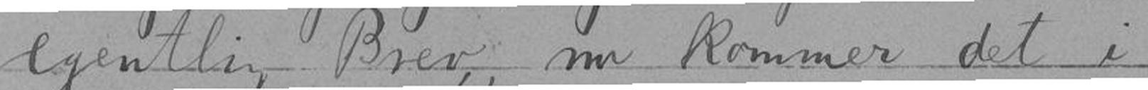egentlig Brev, nu kommer det i
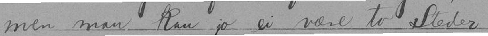men man kan jo ei være to Steder
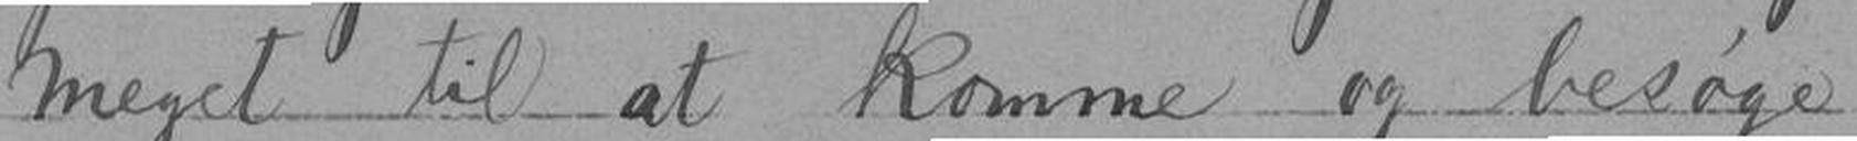meget til at komme og besøge
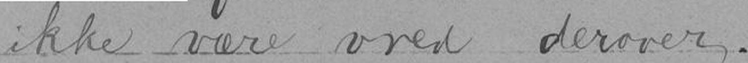ikke værre vred derover.
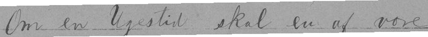Om en Ugestid skal en af vore
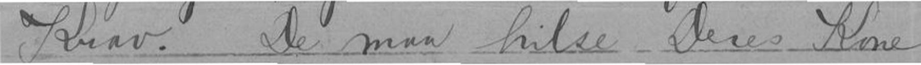Krav. De maa hilse Deres Kone
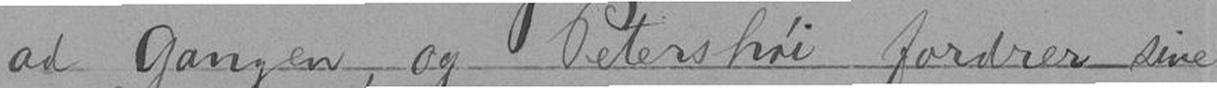ad Gangen, og Petershøi fordrer sine
